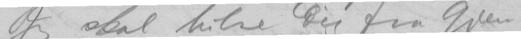Jeg skal hilse Dig fra Gjen-
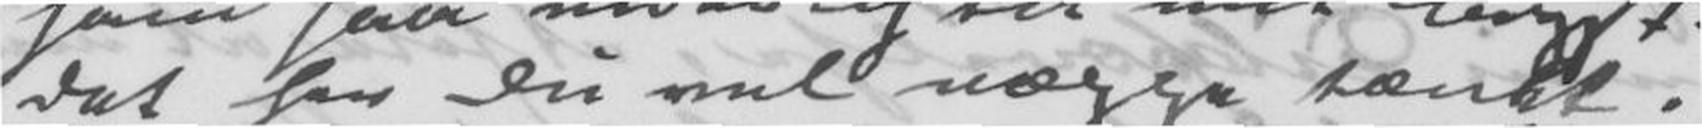Det har Du vel næppe tænkt.
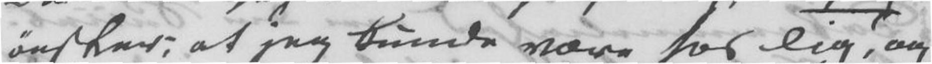ønsker; at jeg kunde være for Dig, og
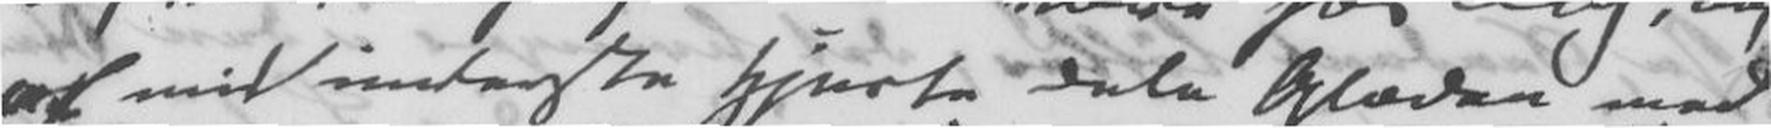af mit inderste hjerte Dele Glæder med
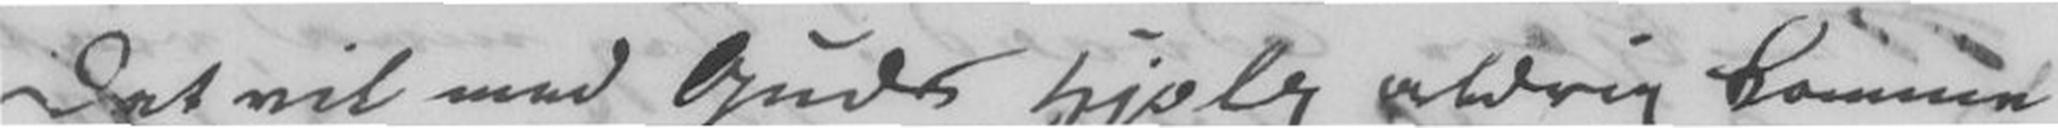Det vil med Guds hjælp aldrig komme
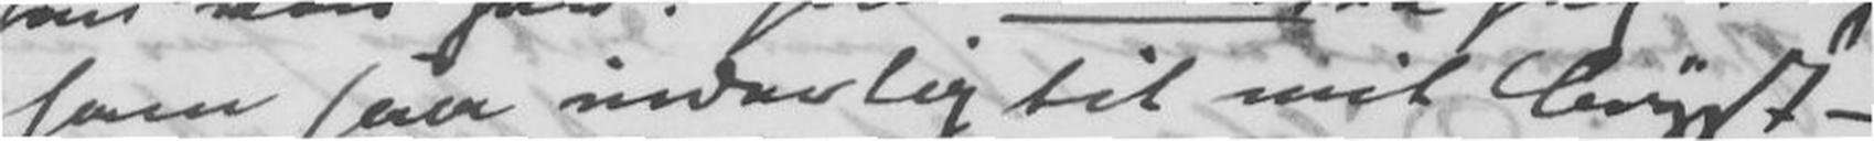ham saa inderlig til mit bryst -
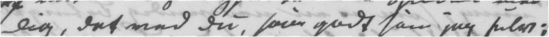Dig, det ved Du, som godt som jeg selv;
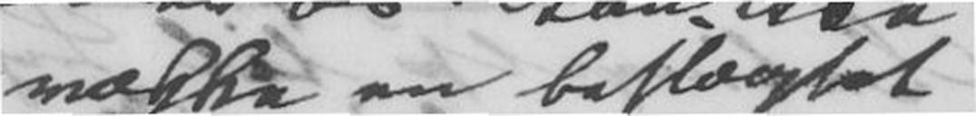række en beslægtet
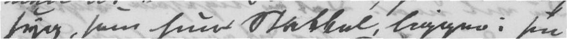syg, som hun Stakkel, ligger i sin
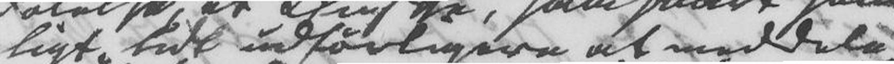ligt lidt udførligere at meddele
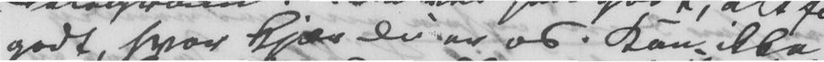godt, hvor Kjær Du er os. Kan ikke
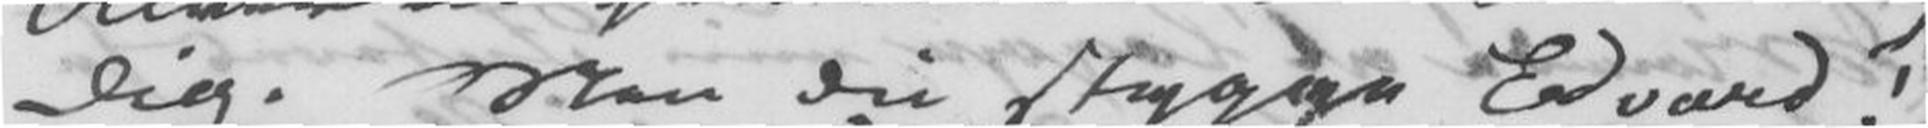Dig. Men Du stygge Edvard!
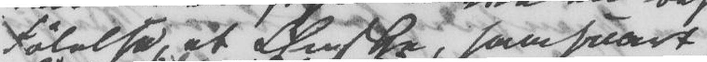følelse, at Huske, ham snart
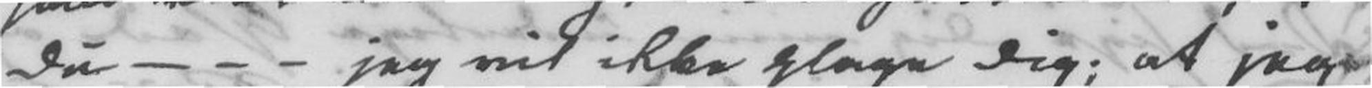Du - - - jeg vil ikke plage Dig; at jeg
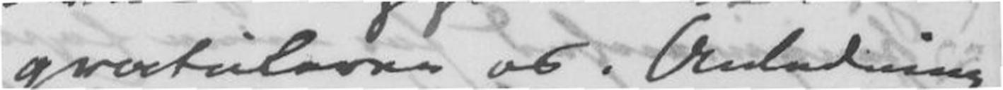gratulere os. Anledning
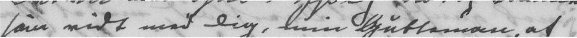som vidt med Dig, min Gutteman, at
